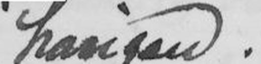haugen.
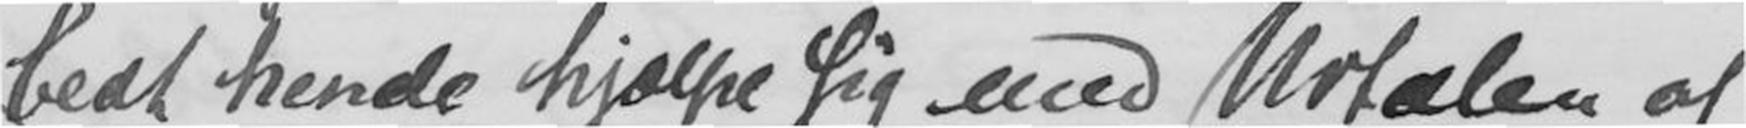bedt hende hjælpe sig med Udtalen af
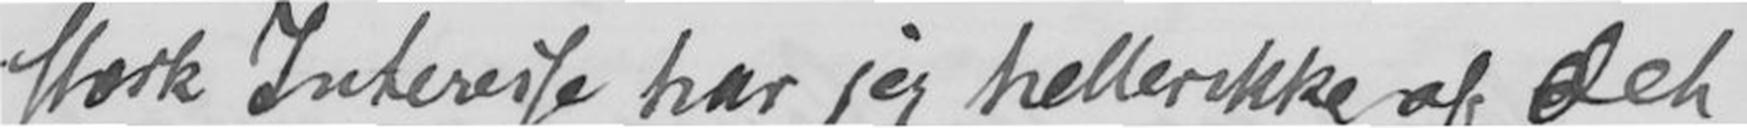stærk Interesse har jeg heller ikke af det,
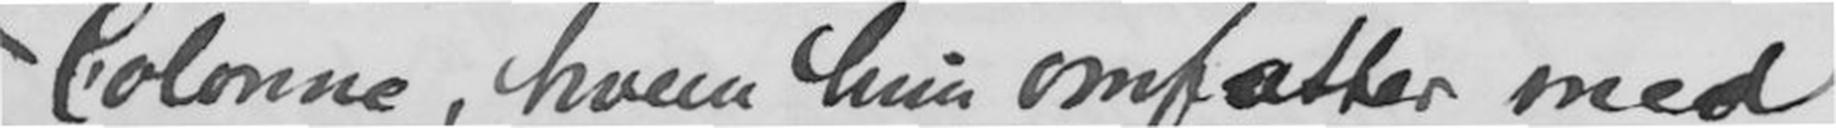Colonne, hvem hun omfatter med
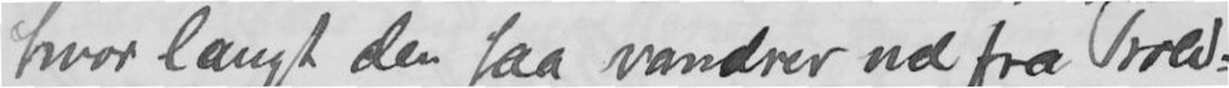hvor langt den saa vandrer ud fra Trold-
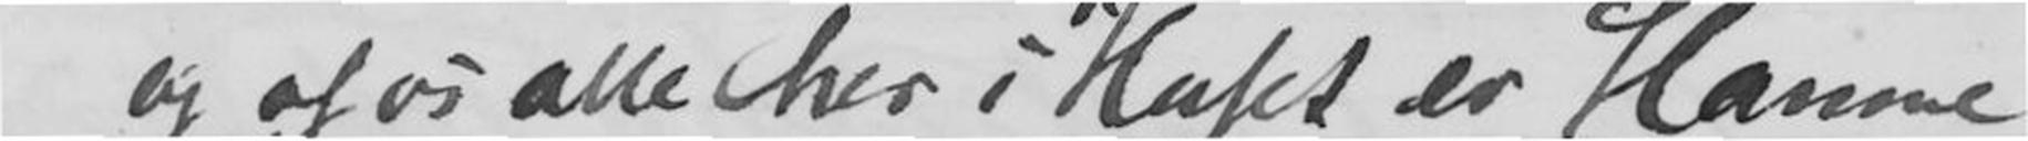og af os alle her i Huset er Hanne
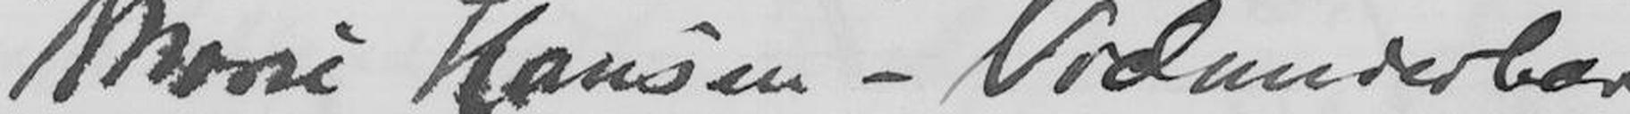Marie Hansen - Vidunderbar
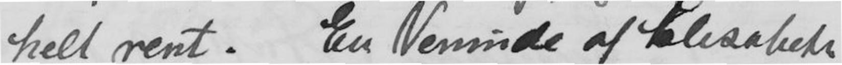helt rent. En Veninde af Elisabeth
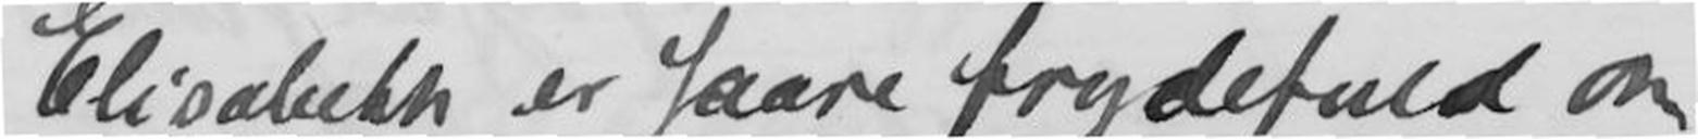Elisabeth er saare frydefuld om
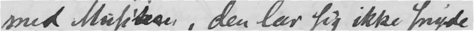med Musiken, den lar sig ikke snyde
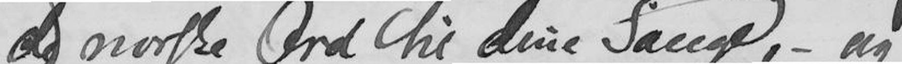de norske Ord til dine Sange, - og
